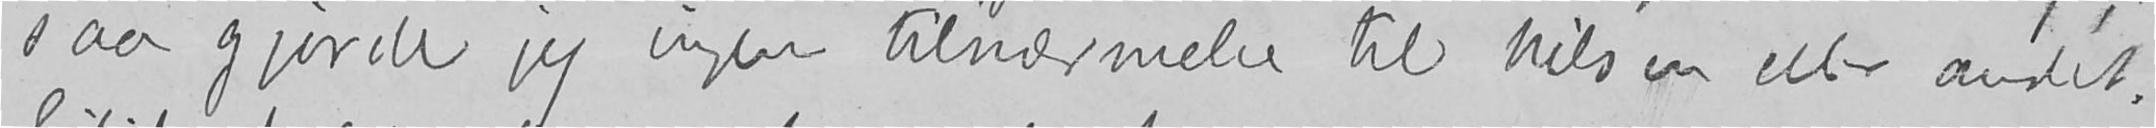saa gjorde jeg ingen tilnærmelse til hilsen eller andet.
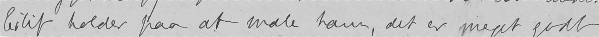Eilif holder paa at male ham, det er meget godt,
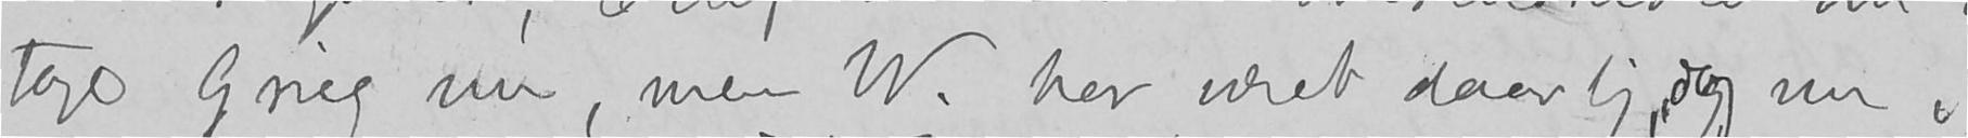tage Grieg nu, men W. har været daarlig, dog nu i
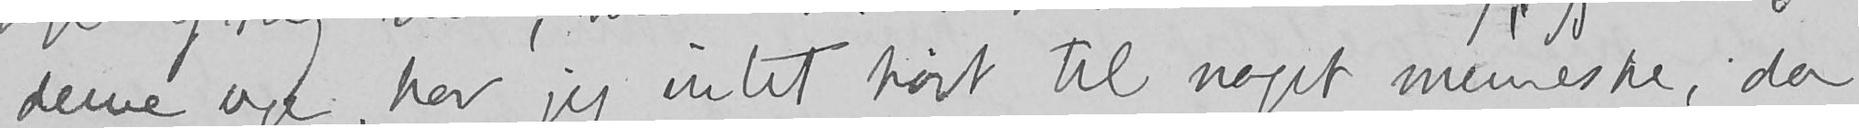denne uge, har jeg intet hørt til noget menneske, da
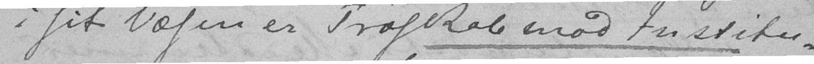i sit Væsen er Troskab mod Institu-
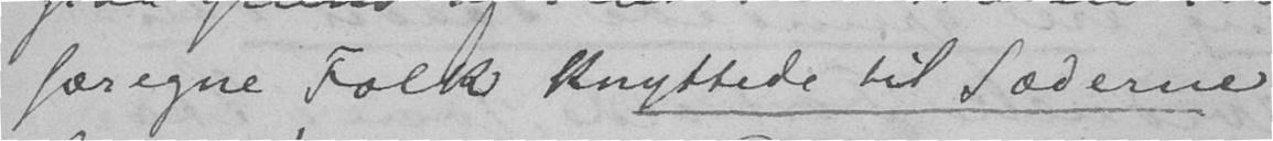særegne Folk knyttede til Sæderne,
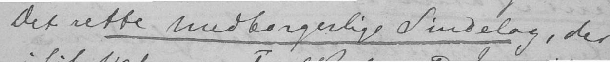Det rette medborgerlige Sindelag, der
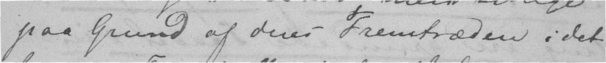paa Grund af dens Fremtræden i det
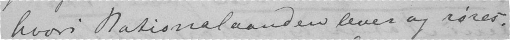hvori Nationalaanden lever og røres.
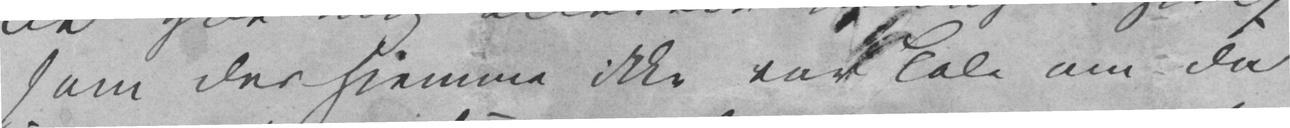som der Hjemme ikke var Tale om da
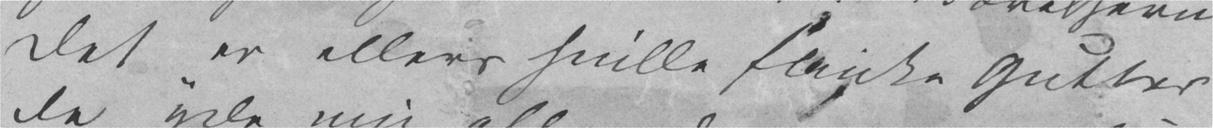Det er ellers snille flinke Gutter,
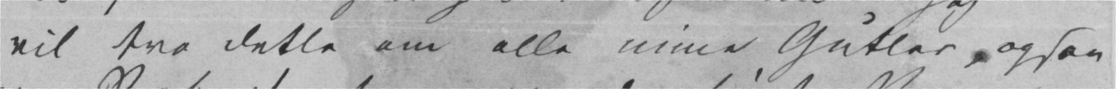vil tro dette om alle mine Gutter, ogsaa
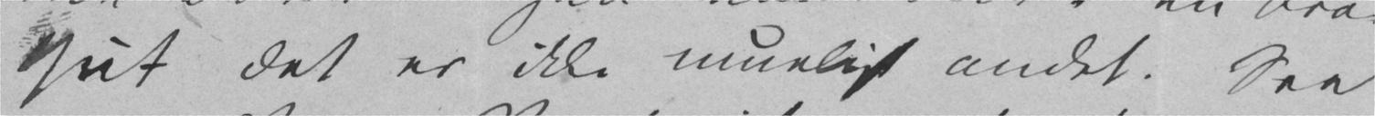Gut det er ikke mueligt andet. See
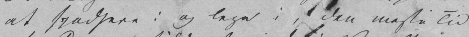at spadsere i og lege i, den meste Tid
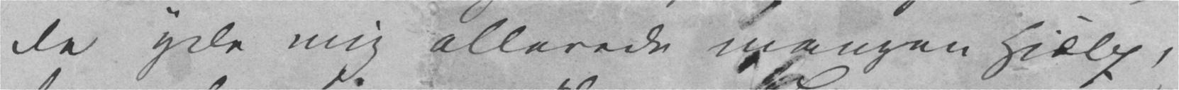de yde mig allerede mangen Hjælp,
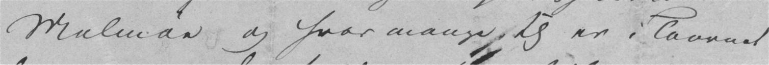Malmøe og hvor mange Kl er i Taarnet
